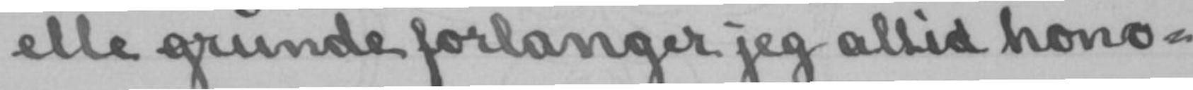elle grunde forlanger jeg altid hono-
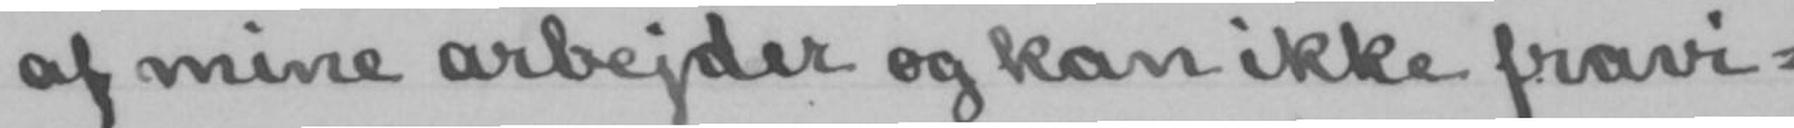af mine arbejder og kan ikke fravi-
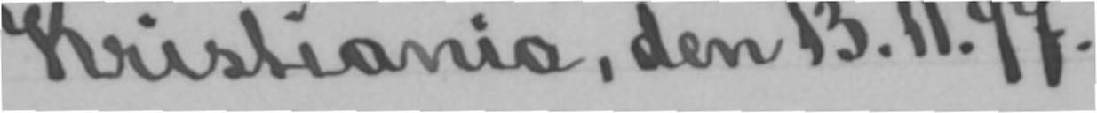Kristiania, den 13.11.97.
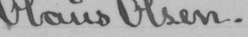Olaus Olsen.
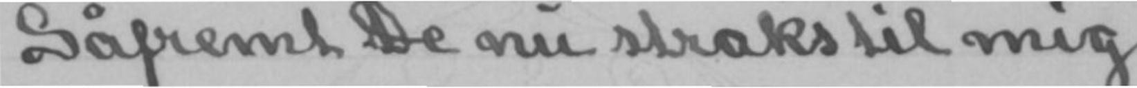Såfremt De nu straks til mig
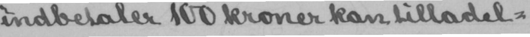indbetaler 100 kroner kan tilladel-
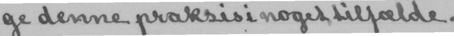ge denne praksis i noget tilfælde.
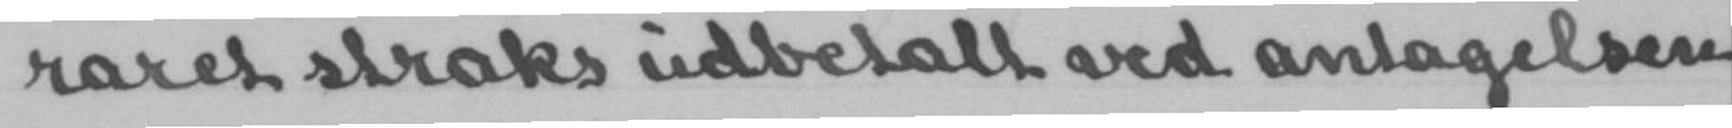raret straks udbetalt ved antagelsen
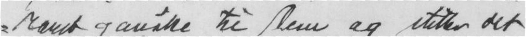mand ganske til Dem og det
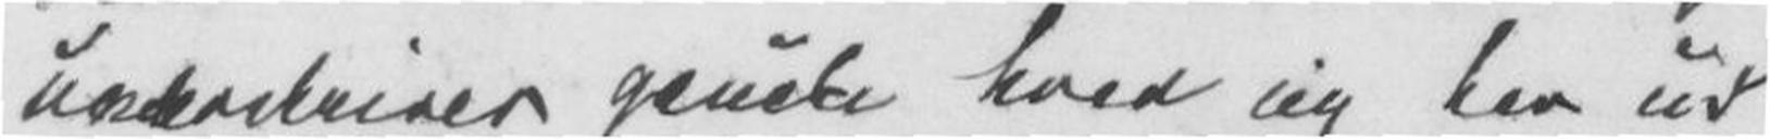ganske hvad jeg har ud-
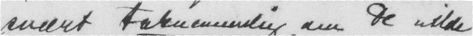svært taknemmlig, om De vilde
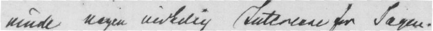minde nogen virkelig for Sagen.
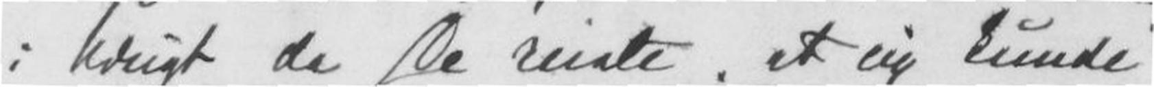kdrigt da De reiste, at jeg kunde
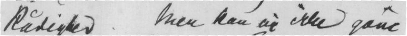Rådighed. Mer kan jeg ikke give.
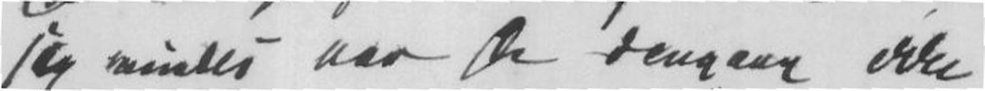jeg mindes har saa De dengang ikke
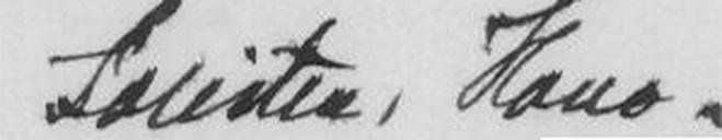Solisten, Haus-
